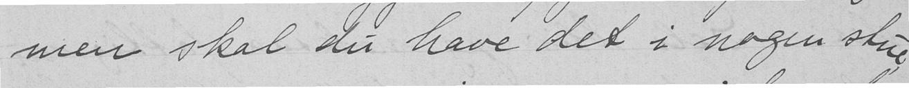men skal du have det i nogen stue
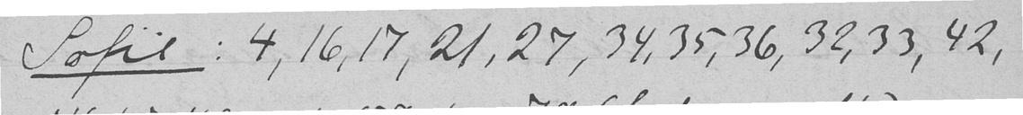Sofie: 4, 16, 17, 21, 27, 34, 35, 36, 32, 33, 42,
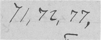71, 72, 77,
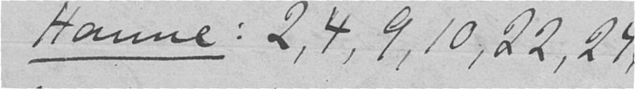Hanne: 2, 4, 9, 10, 22, 24
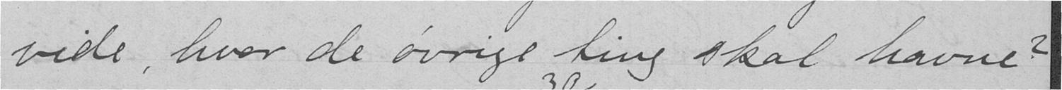vide, hvor de øvrige ting skal havne?
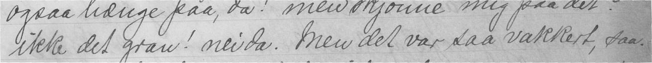ikke det gran! nei da. Men det var saa vakkert, saa.
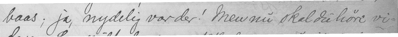baas; ja, nydelig var der! Men nu skal du høre vi-
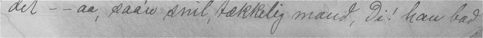det - - aa, saa'n snil, tækkelig mand, Di! han bad
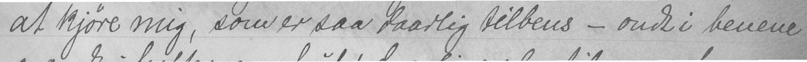at kjøre mig, som er saa daarlig tilbens - ondt i benene
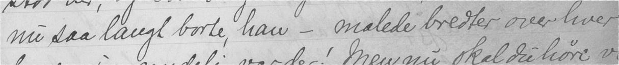nu saa langt borte, han - malede bredter over hver
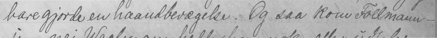bare gjorde en haandbevægelse. Og saa kom Follmann -
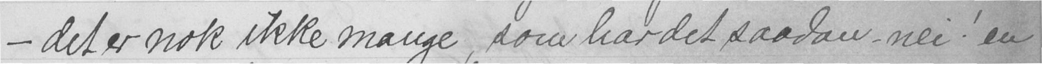- det er nok ikke mange, som har det saadan - nei! en
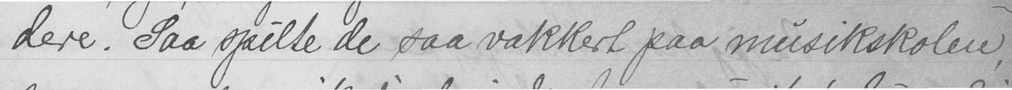dere. Saa spilte de saa vakkert paa musikskolen,
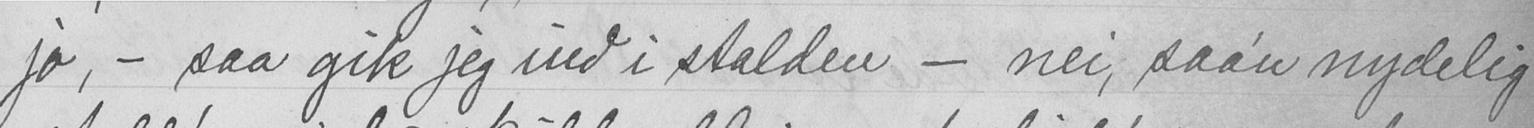jo, - saa gik jeg ind i stalden - nei, saa'n nydelig
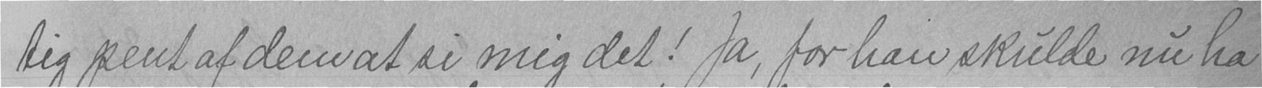tig pent af dem at si mig det! Ja, for han skulde nu ha
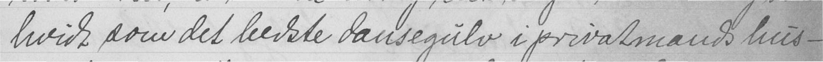hvidt som det bedste dansegulv i privatmands hus -
